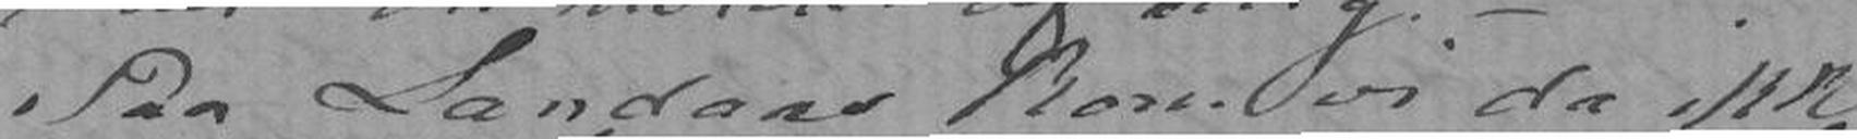Paa Landaas kom vi da ikke
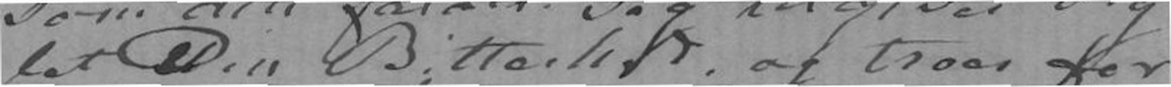let Din Bitterhed, og troer for
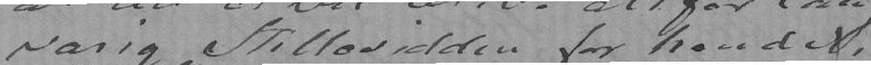varig Stillesidden for hende,
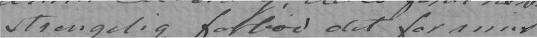strengelig forbød det for min
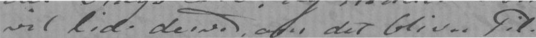vil lide derved, om det bliver Til-
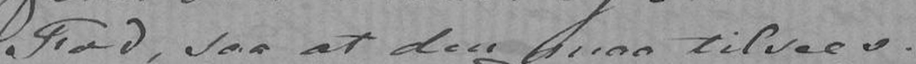Fod, saa at den maa tilsees.
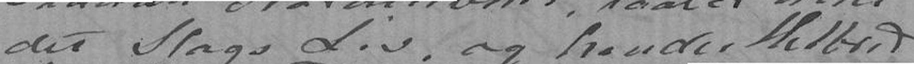det Slags Liv, og hendes Helbred
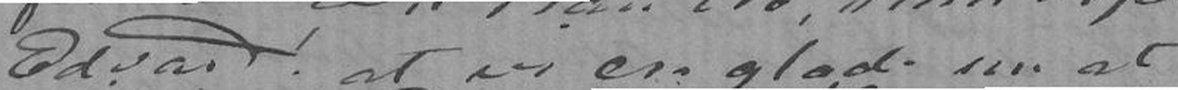Edvard! at vi ere glad nu at
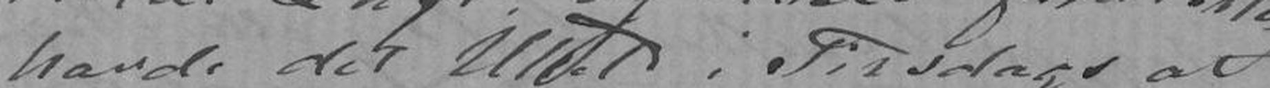havde det Uheld i Tirsdag at
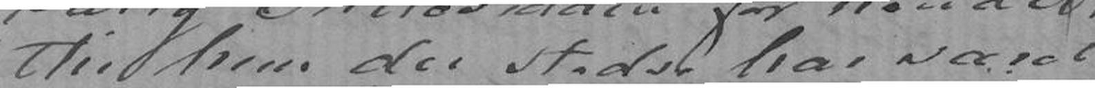thi hun der stedse har været
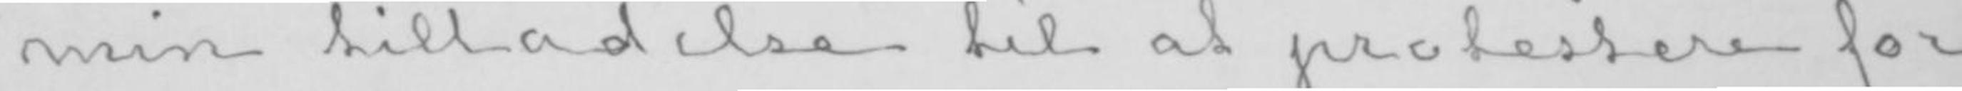min tilladelse til at protestere for
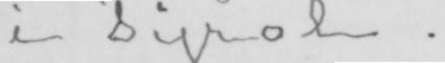i Tyrol.
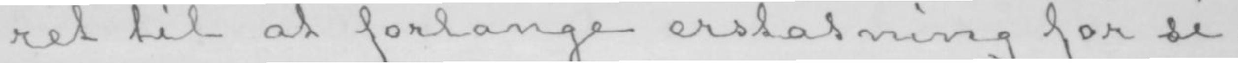ret til at forlange erstatning for si-
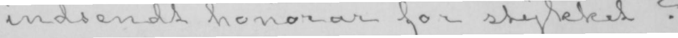indsendt honorar for stykket?
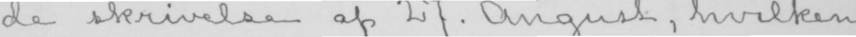de skrivelse af 27. August, hvilken
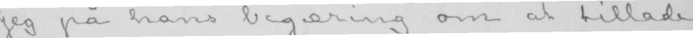jeg på hans begæring om at tillade
